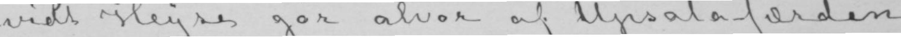vidt Heyse gør alvor af Upsalafærden
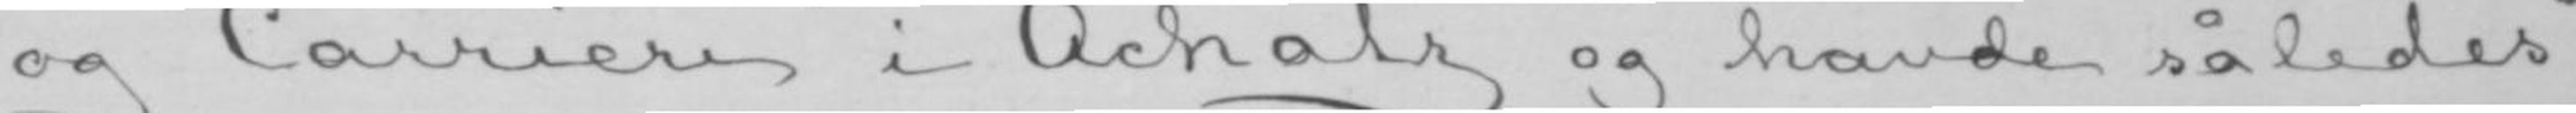og Carriere i Achatz og havde således
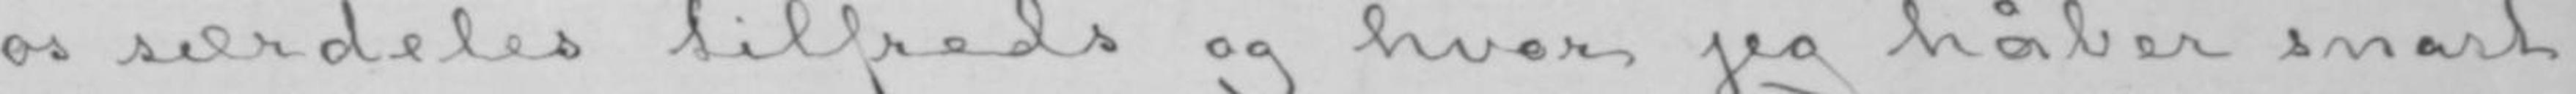os særdeles tilfreds og hvor jeg håber snart
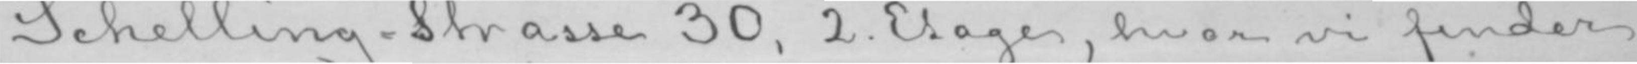Schelling-Strasse 30, 2. Etage, hvor vi finder
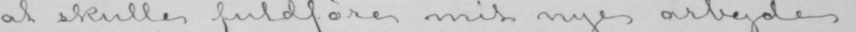at skulle fuldføre mit nye arbejde.
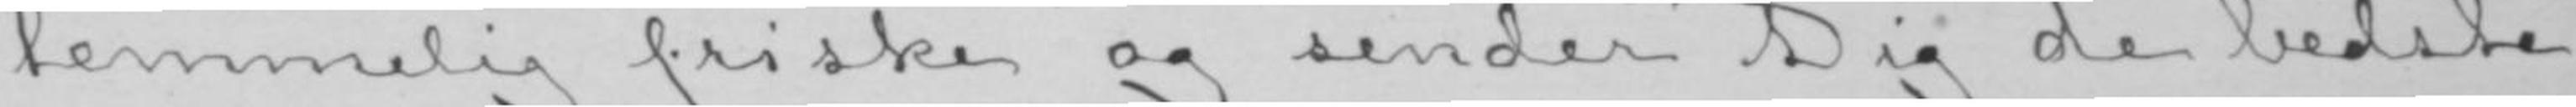temmelig friske og sender Dig de bedste
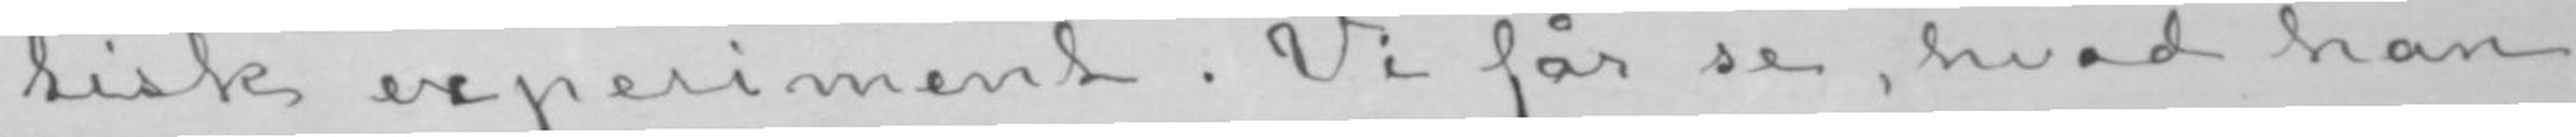tisk experiment. Vi får se, hvad han
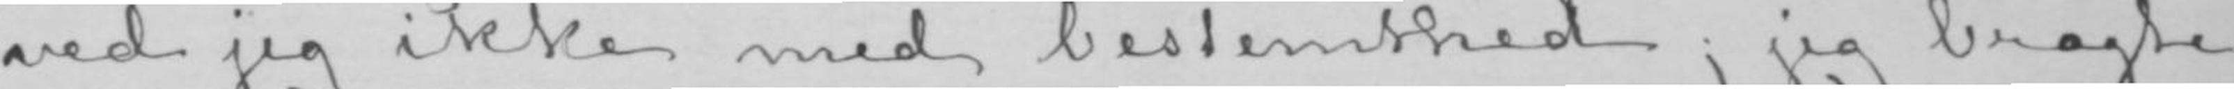ved jeg ikke med bestemthed; jeg bragte
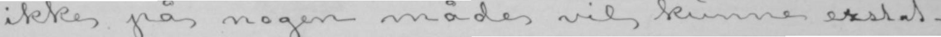ikke på nogen måde vil kunne erstat-
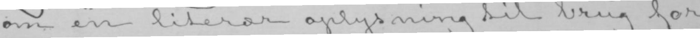om en literær oplysning til brug for
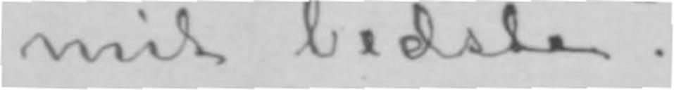mit bedste.
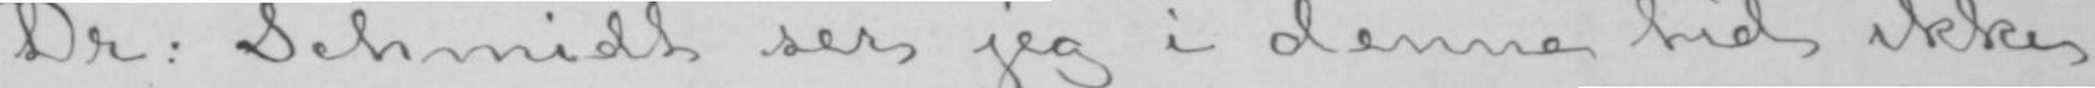Dr: Schmidt ser jeg i denne tid ikke
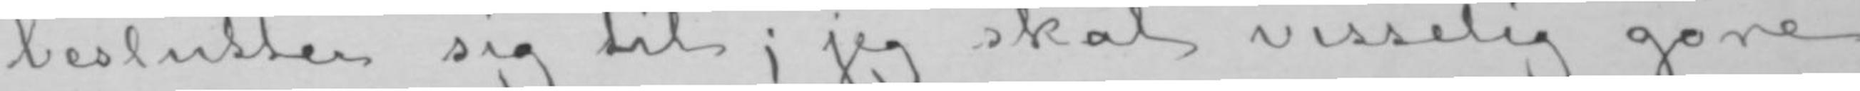beslutter sig til; jeg skal visselig gøre
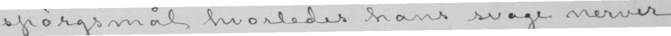spørgsmål hvorledes hans svage nerver
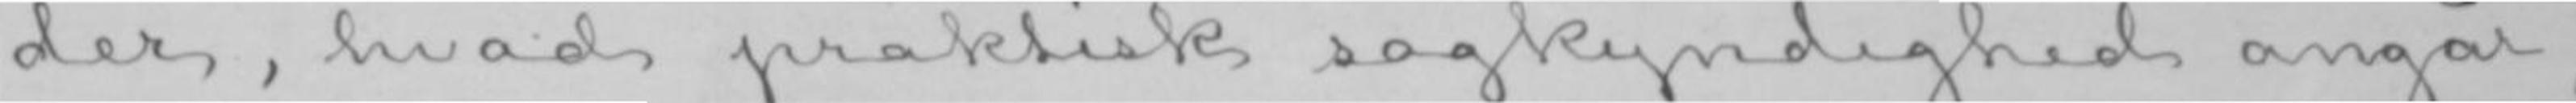der, hvad praktisk sagkyndighed angår,
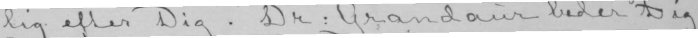lig efter Dig. Dr: Grandaur beder Dig
